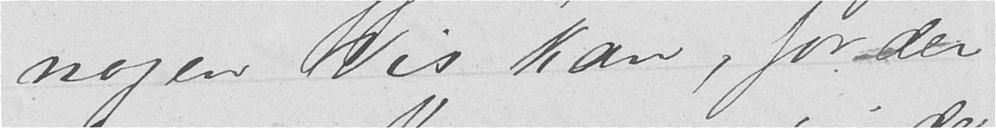nogen Vis kan, for der
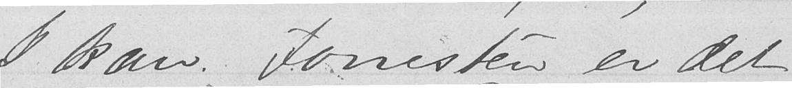I kan. Forresten er det
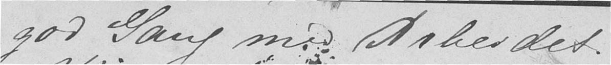god Gang med Arbeidet.
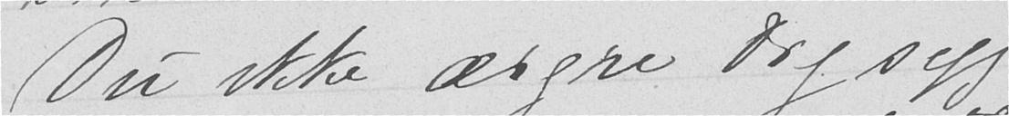Du ikke ærgre dig syg
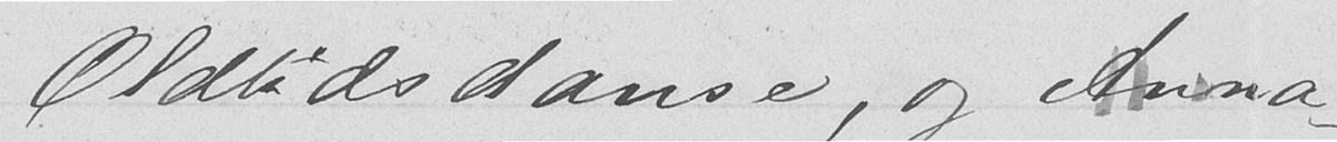Oldtidsdanse, og Anna-
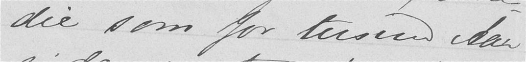die som for tusind Aar
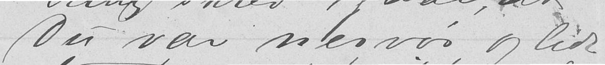Du var nervøs og lidt
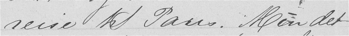reise til Paris. Min det
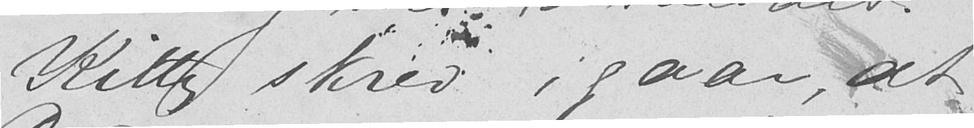Kitty skrev i gaar, at
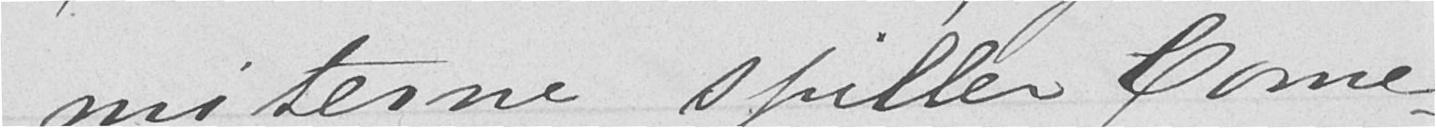miterne spiller Come-
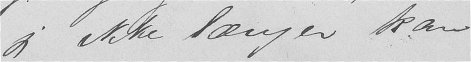jeg ikke længer kan
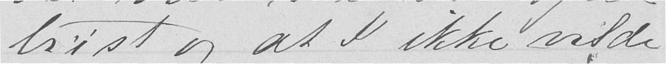trist og at I ikke vilde
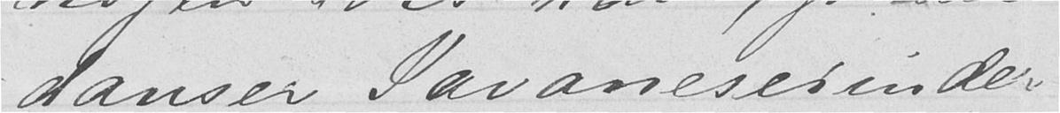danser Javaneserinder
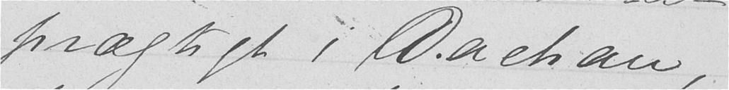prægtigt i Dachan,
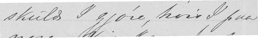skulde I gjøre, hvis I paa
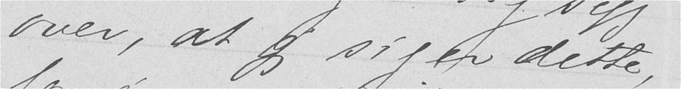over, at jeg siger dette
